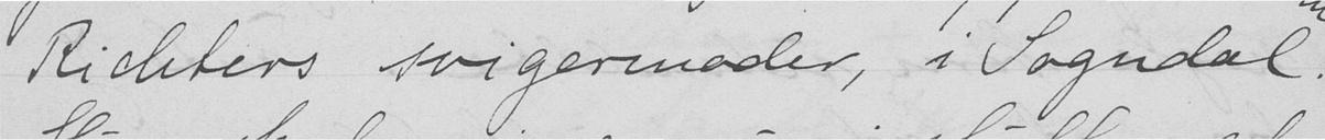Richters svigermoder, i Sogndal.
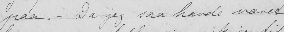paa. - Da jeg saa havde været
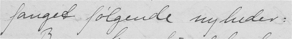fanget følgende nyheder:
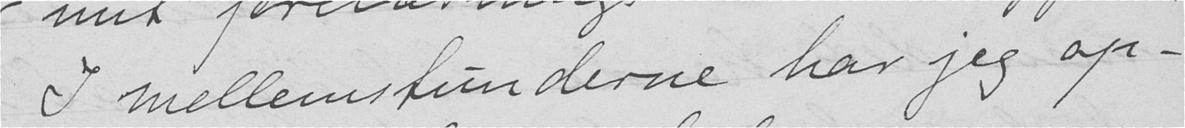I mellemstunderne har jeg op-
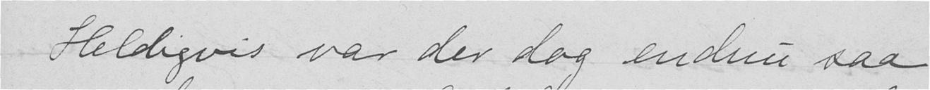Heldigvis var der dog endnu saa
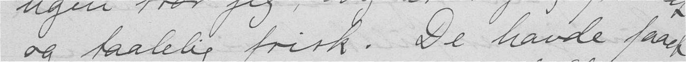og taalelig frisk. De havde faaet
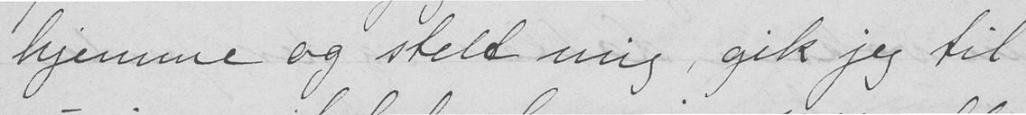hjemme og stelt mig, gik jeg til
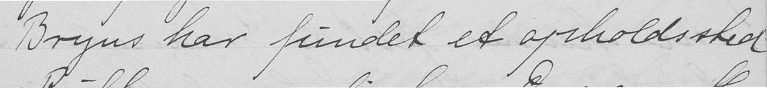Bryns har fundet et opholdssted
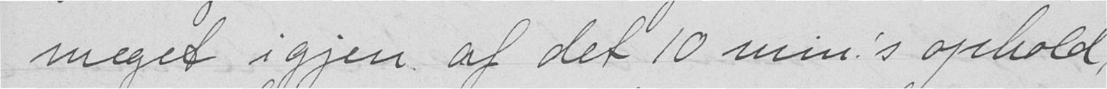meget igjen af det 10 min.'s ophold,
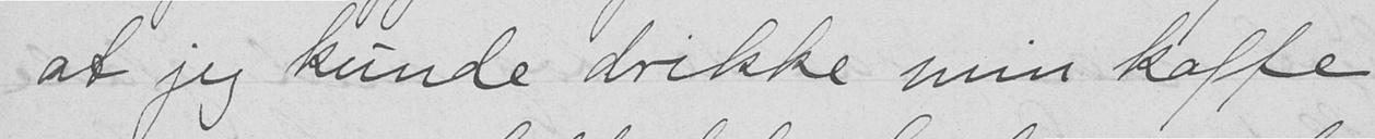at jeg kunde drikke min kaffe
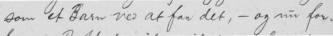som et Barn ved at faa det, - og nu for-
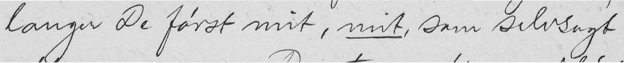langer De først mit, mit, som selvsagt
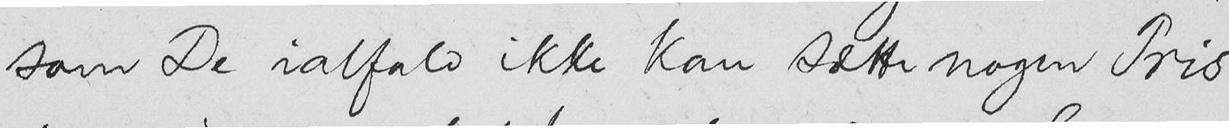som De ialfald ikke kan sætte nogen Pris
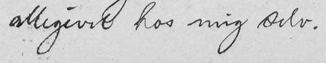alligevel hos mig selv.
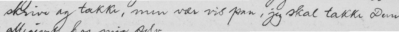skrive og takke, men vær vis paa, jeg skal takke Dem
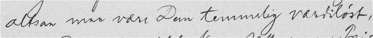altsaa maa være Dem temmelig værdiløst,
